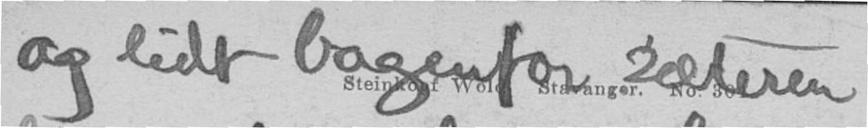og lidt bagenfor sæteren
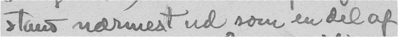stand nærmest ud som en del af
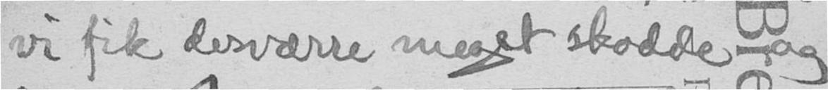vi fik desværre meget skodde, - og
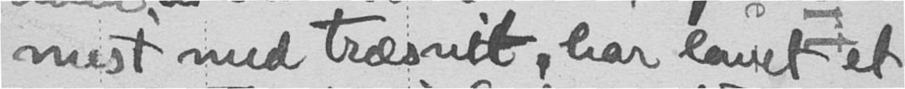mest med træsnit, har lavet et
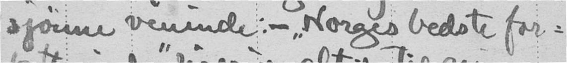sjønne veninde: - "Norges bedste for-
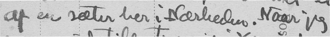af en sæter her i Nærheden. Naar jeg
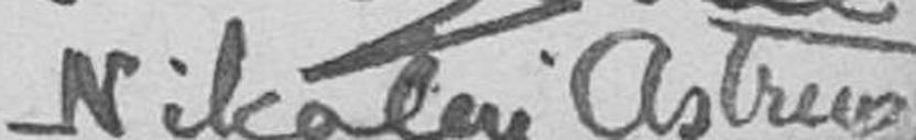Nikolai Astrup
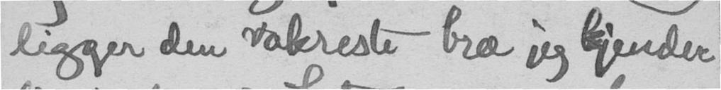ligger den vakreste bræ jeg kjender
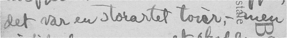det var en storartet touer, - men
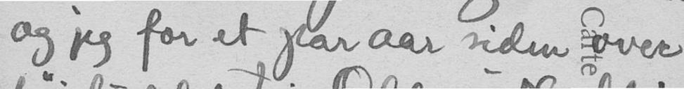og jeg for et par aar siden over
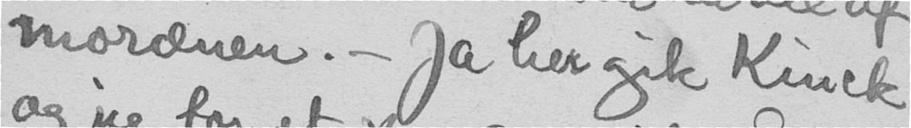morænen. - Ja her gik Kinck
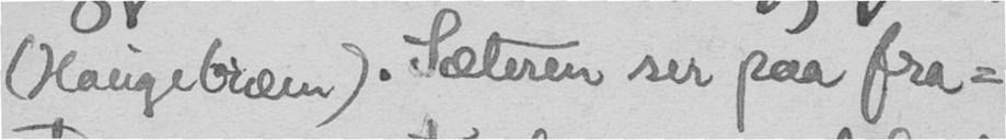(Haugebræen). Sæteren ser paa fra-
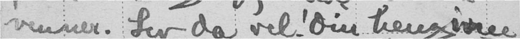venner, Lev da vel! din hengivne
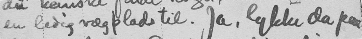en ledig vægplads til. Ja, lykke da paa
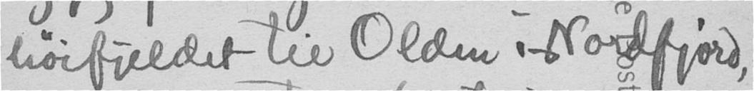høifjeldet til Olden i Nordfjord, -
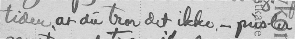tiden, at du tror det ikke - puster
